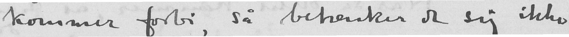kommer forbi, så betænker de sig ikke
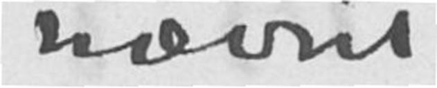nævnt
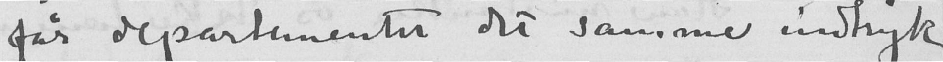får departementet det samme indtryk
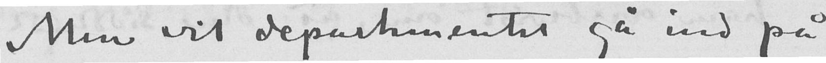Men vil departementet gå ind på
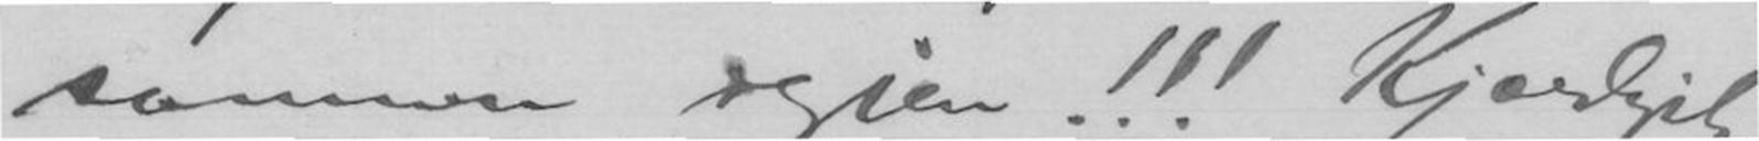sammen igjen!!! Kjærligste
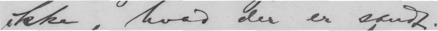ikke, hvad der er sandt.
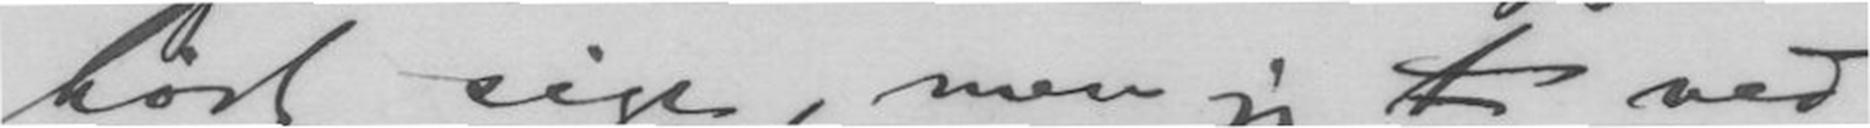hørt sige, men jeg t ved
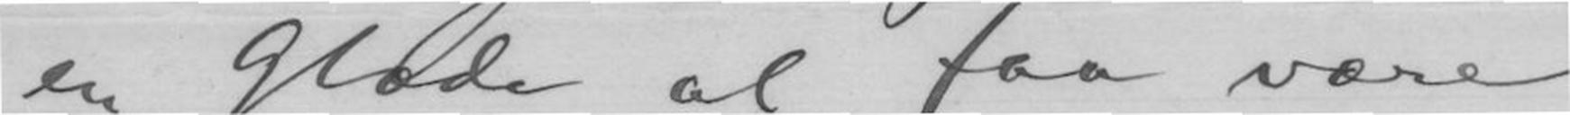en Glæde at faa være
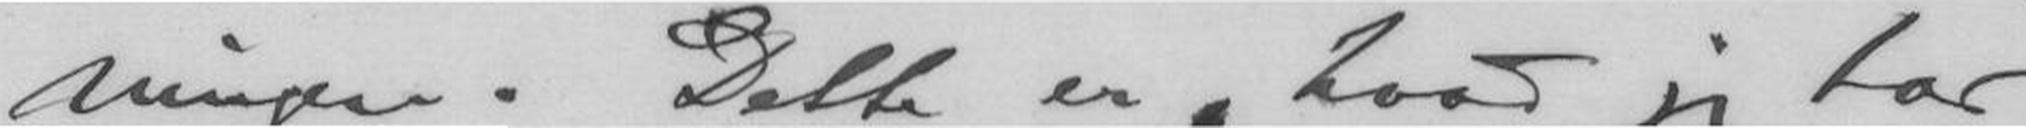ningen. Dette er hvad jeg har
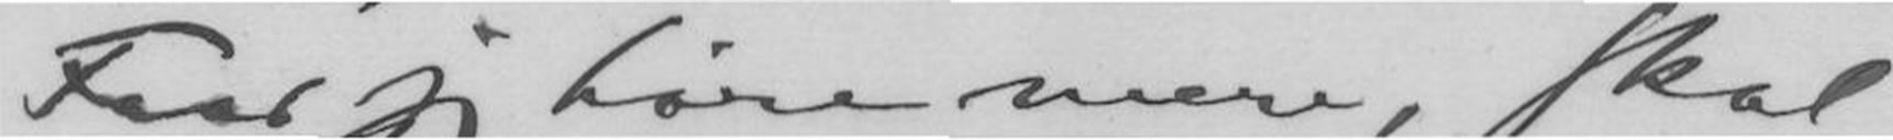Faar jeg høre mere, skal
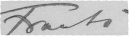Frants
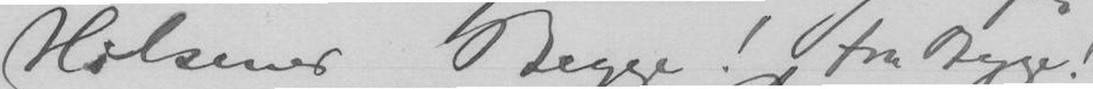Hilsener Begge! fra Begge!
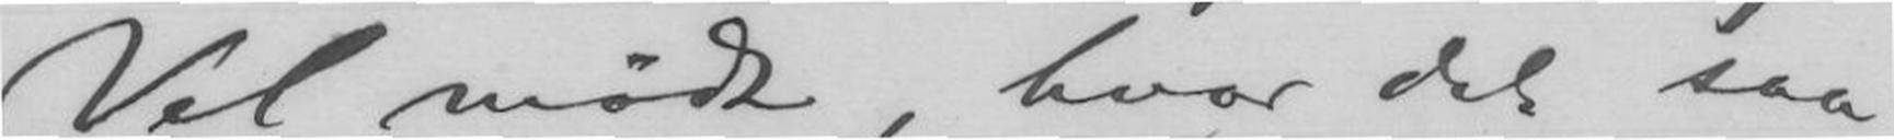Vel mødt, hvor det saa
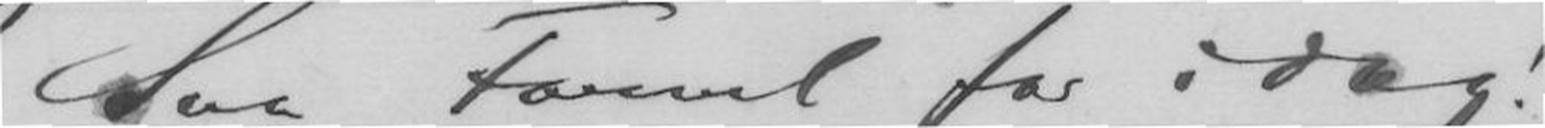Saa Farvel for idag!
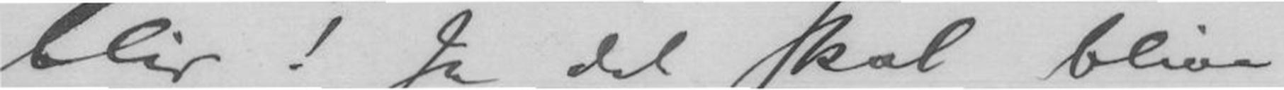blir! Ja det skal blive
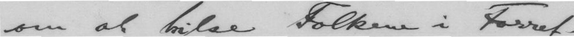om at hilse Folkene i Forret-
